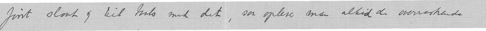først slaat sig til taals med det, saa oplever man altid de overraskende
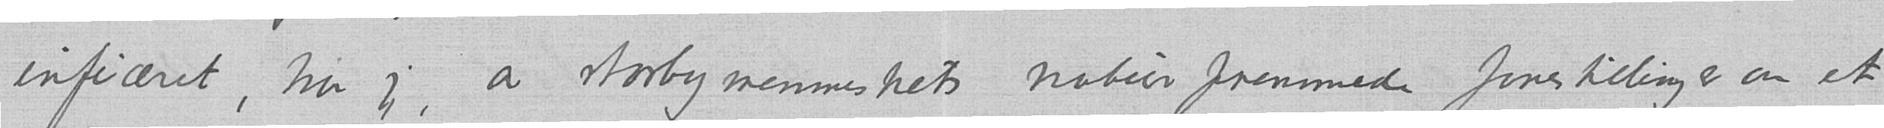inficeret, tror jeg, av storbymenneskets naturfremmede forestillinger om et
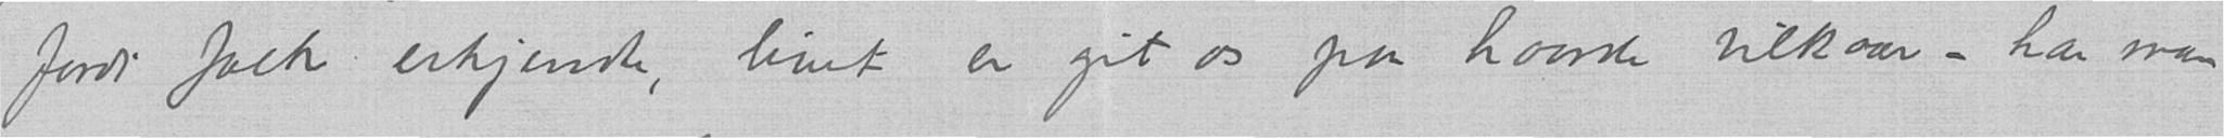fordi folk erkjendte, livet er git os paa haarde vilkaar – har man
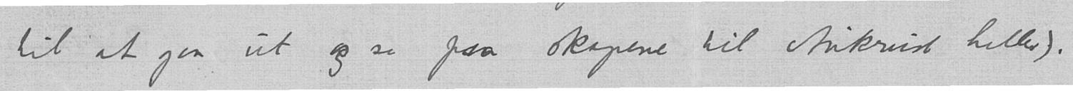til at gaa ut og se paa skapene til Aukrust heller).
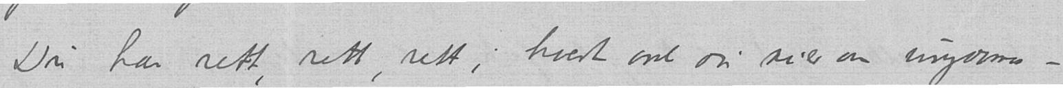Du har rett, rett, rett, hvert ord du sier om ungdoms-
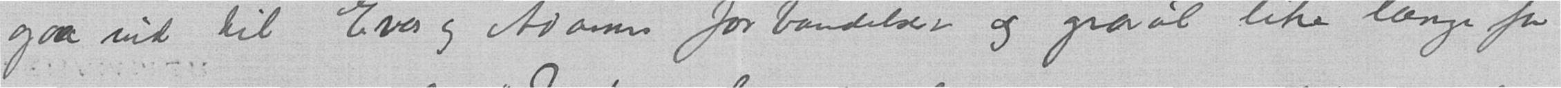gaa ind til Evas og Adams forbandelser og gravøl like længe for
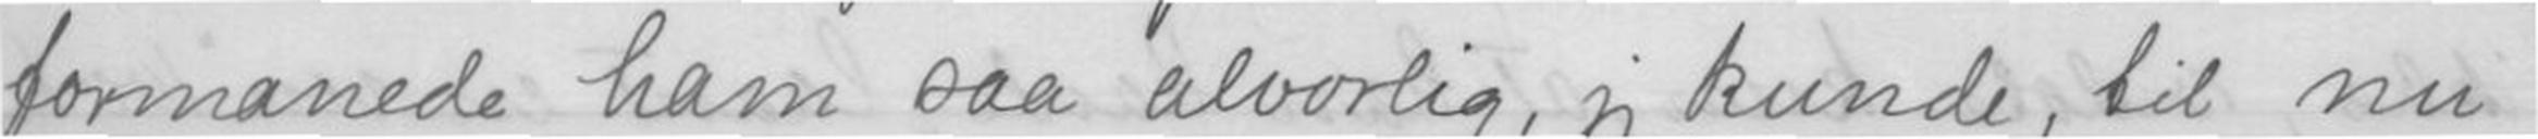formanede ham saa alvorlig, jeg kunde, til nu
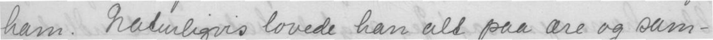ham. Naturligvis lovede han alt paa ære og sam-
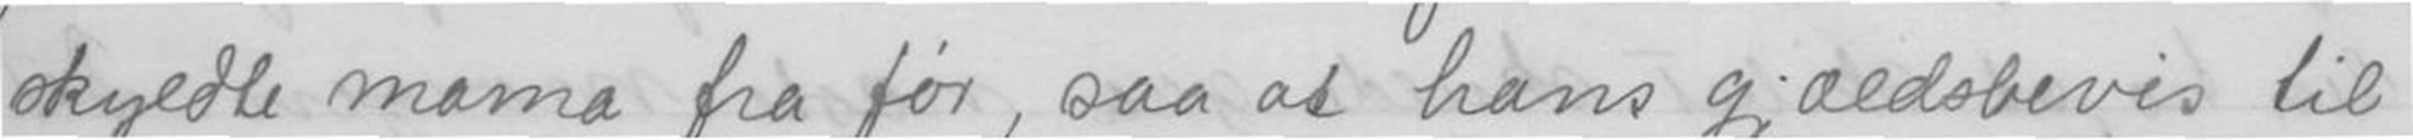skyldte mama fra før, saa at hans gjældsbevis til
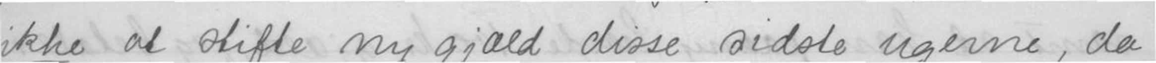ikke at stifte ny gjæld disse sidste ugerne, da
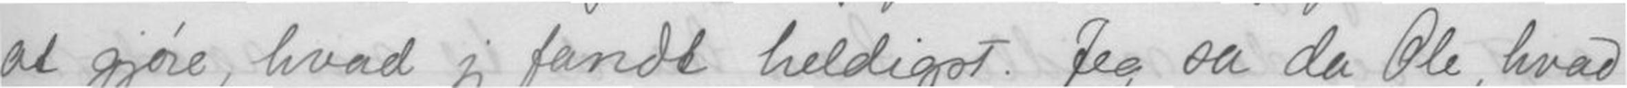at gjøre, hvad jeg fandt heldigst. Jeg sa da Ole, hvad
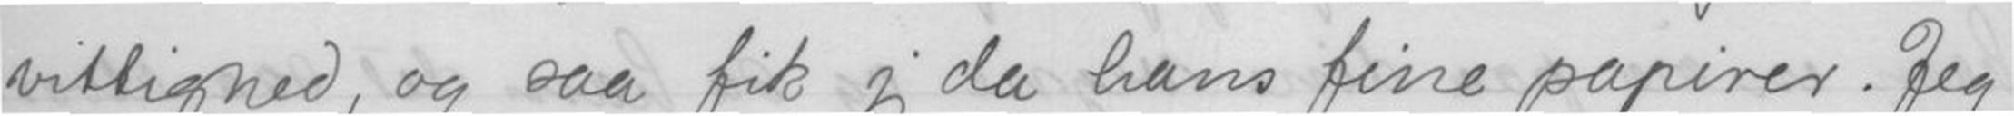vittighed, og saa fik jeg da hans fine papirer. Jeg
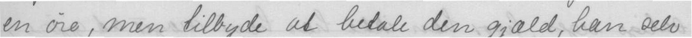en øre, men tilbyde at betale den gjæld, han selv
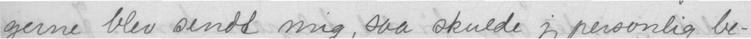gerne blev sendt mig, saa skulde jeg personlig be-
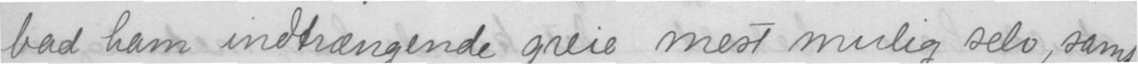bad ham indtrængende greie mest mulig selv, samt
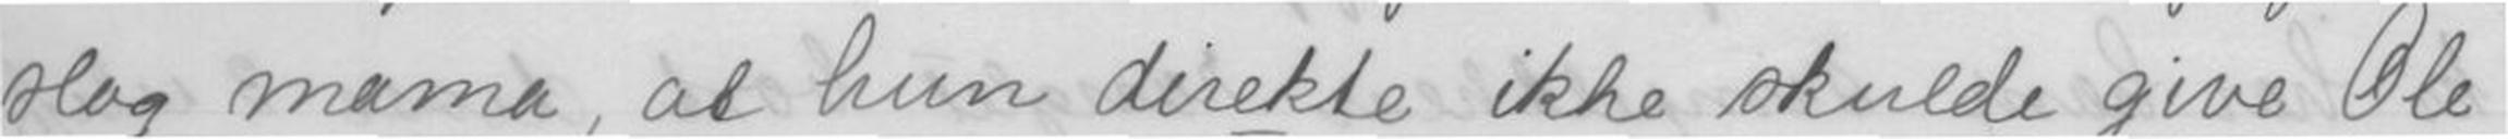slog mama, at hun direkte ikke skulde give Ole
